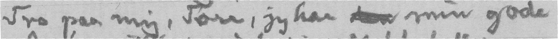Tro paa mig, Tore, jeg har den min gode
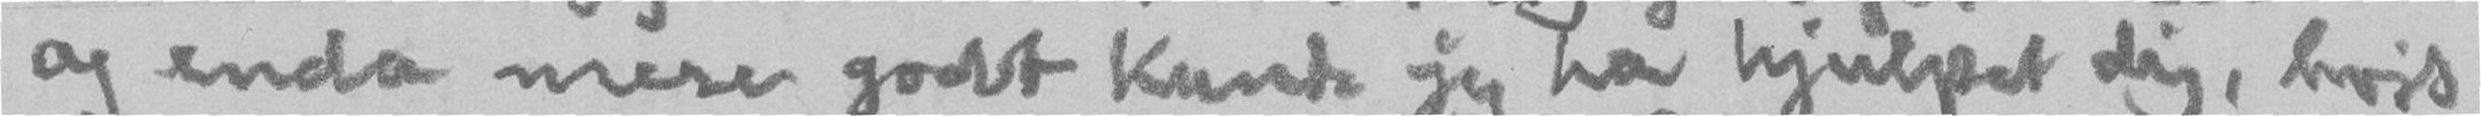og enda mere godt kunde jeg ha hjulpet dig, hvis
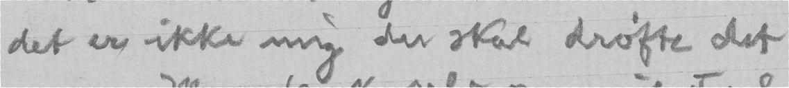det er ikke mig du skal drøfte det
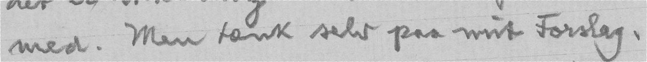med. Men tænk selv paa mit Forslag.
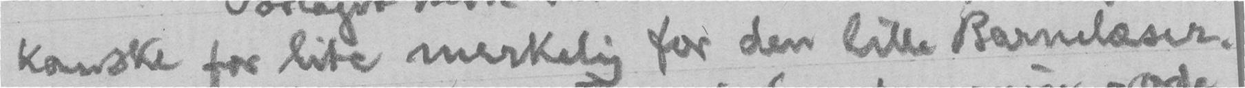kanske for lite merkelig for den lille Barnelæser.
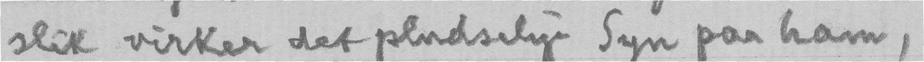slik virker det pludselige Syn paa ham,
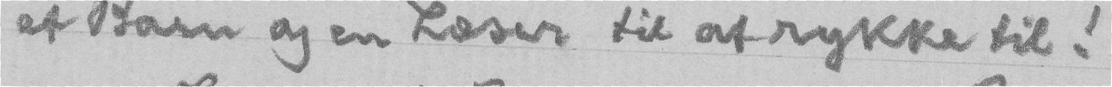et Barn og en Læser til at rykke til!
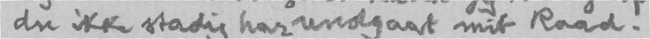du ikke stadig har undgaat mit Raad.
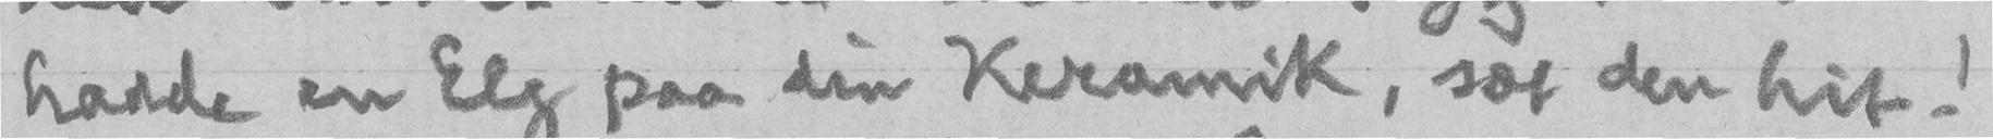hadde en Elg paa din Keramik, sæt den hit!
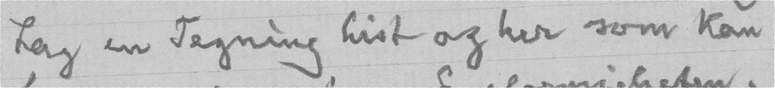Lag en Tegning hist og her som kan
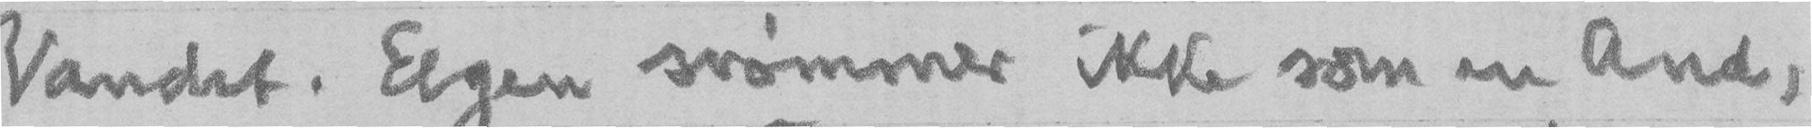Vandet. Elgen svømmer ikke som en And,
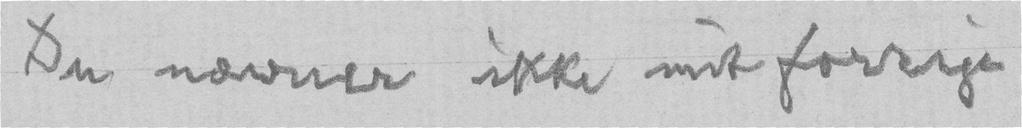Du nævner ikke mit forrige
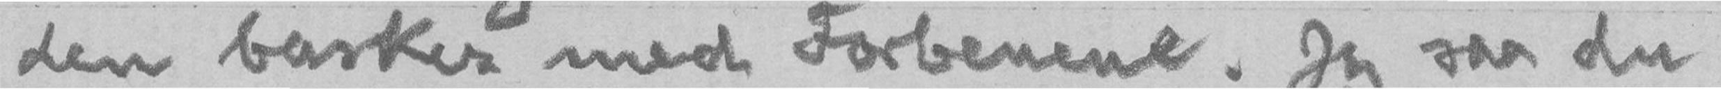den basker med Forbenene. Jeg saa du
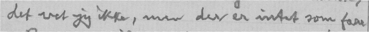det vet jeg ikke, men der er intet som faar
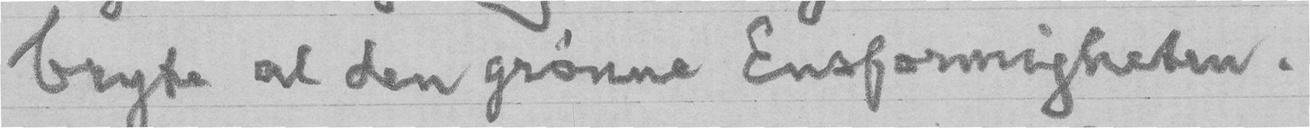bryte al den grønne Ensformigheten.
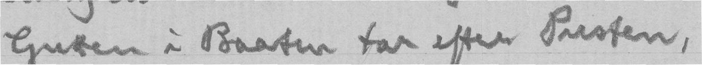Gutten i Baaten tar efter Pusten,
